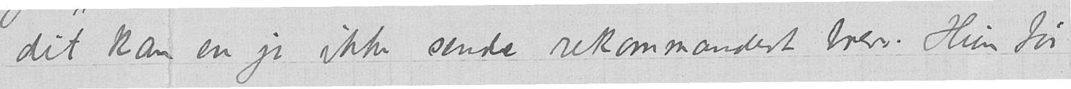dit kan en jo ikke sende rekommandert brev. Hun tør
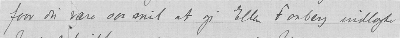faar du være saa snil at gi Ellen Forberg indlagte
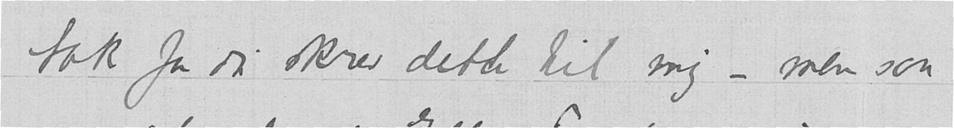tak for du skrev dette til mig – men saa
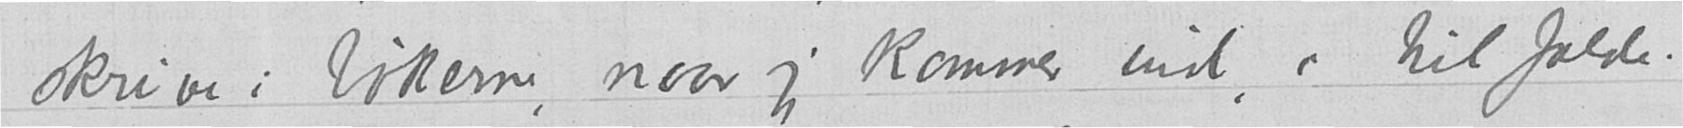skrive i bøkerne, naar jeg kommer ind, i tilfælde.
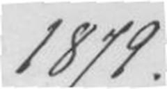1879.
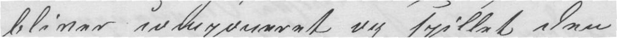bliver imponeret og spillet den
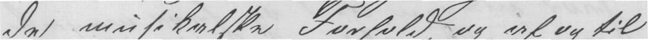de musikalske Forhold og af og til
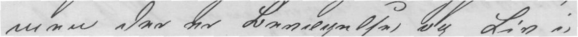men der er Bevægelse og Liv i
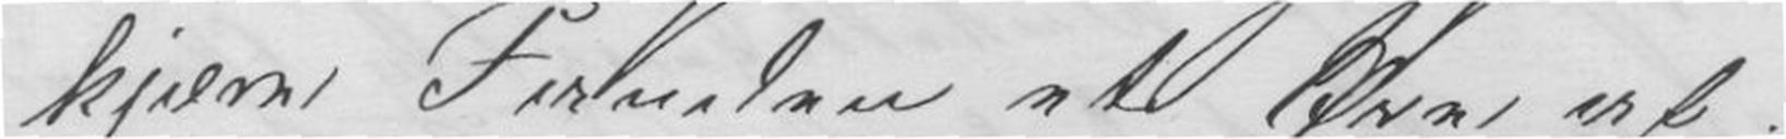kjære Fanden et Øre af!
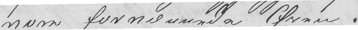vore forvænnede Øren.
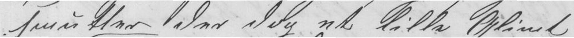smutter der dog et lille Glimt
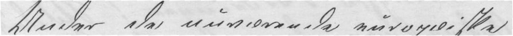Under de nuværende europæiska
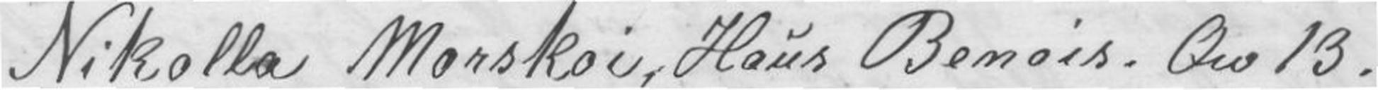Nikolla Morskoi, Haus Benois. Qw 13.
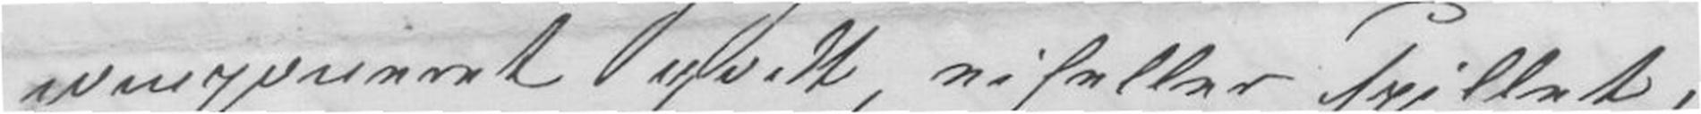imponeret godt eiheller spillet,
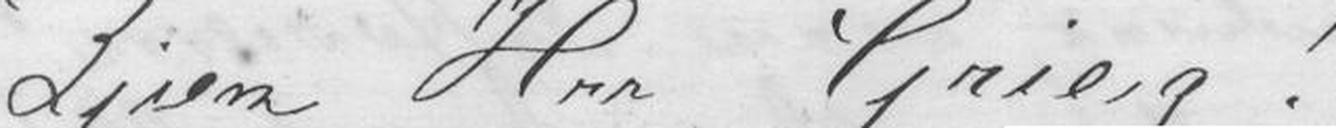Kjære Hrr Grieg!
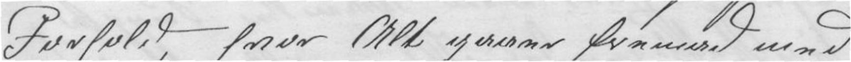Forhold hvor Alt gaaer fremad med
